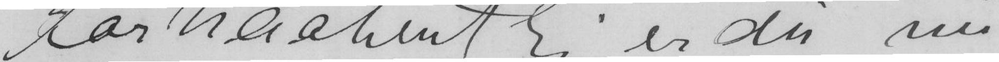Forhaabentlig er du nu
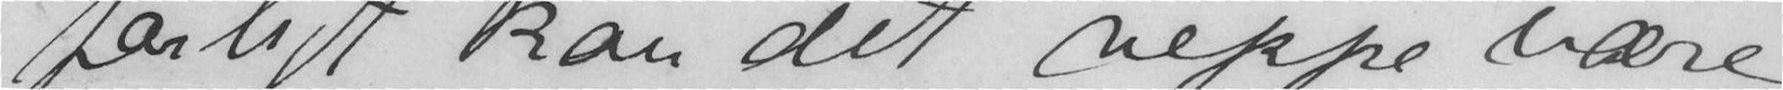farligt kan det neppe være
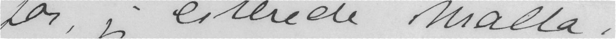for, jeg citerede Malla i
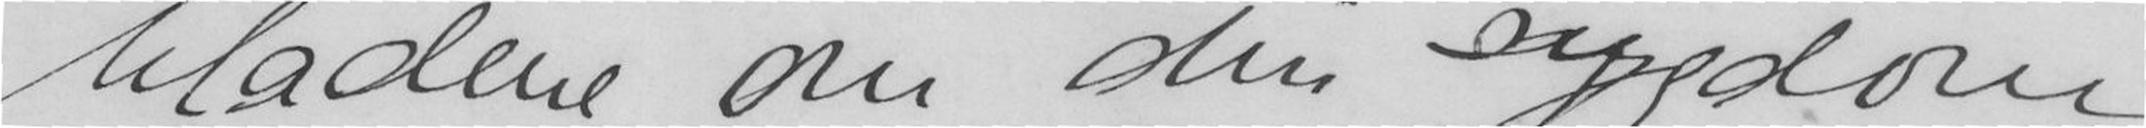bladene om din sygdom
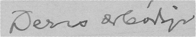Deres ærbødige
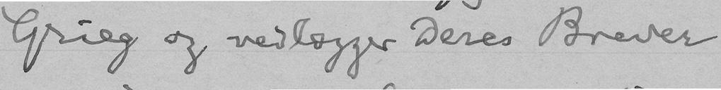Grieg og vedlægger Deres Brever
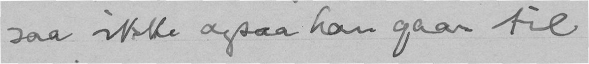saa ikke ogsaa han gaar til
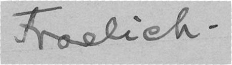Froelich.
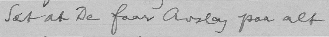Sæt at De faar Avslag paa alt
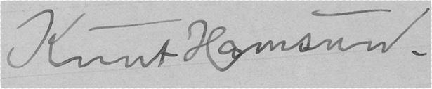Knut Hamsun.
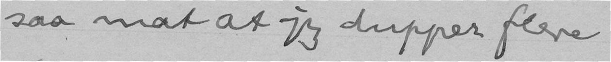saa mat at jeg dupper flere
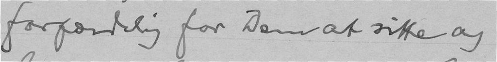forfærdelig for Dem at sitte og
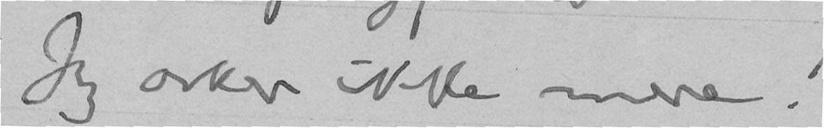Jeg orker ikke mere!
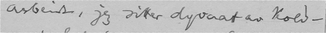arbeide, jeg sitter dyvaat av Kold-
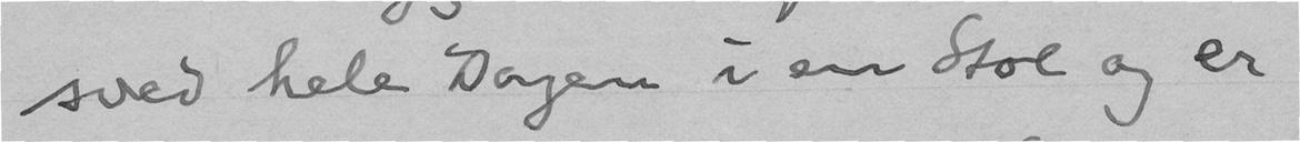sved hele Dagen i en Stol og er
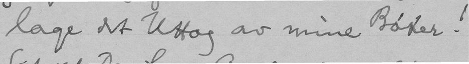lage det Uttog av mine Bøker!
